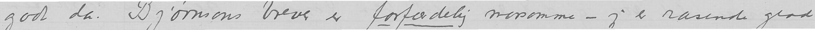godt da. Bjørnsons brever er forfærdelig morsomme – jeg er rasende glad
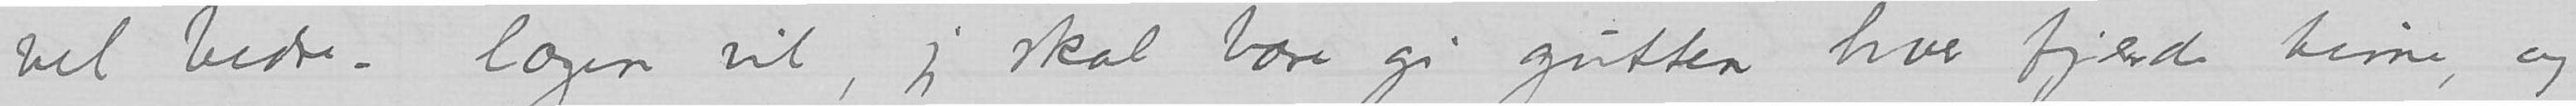vel bedre – lægen vil, jeg skal bare gi gutten hver fjerde time, og
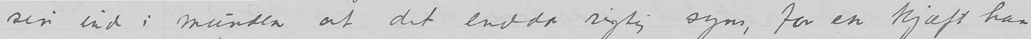sin ind i munden at det endda rigtig syns, for en kjæft han
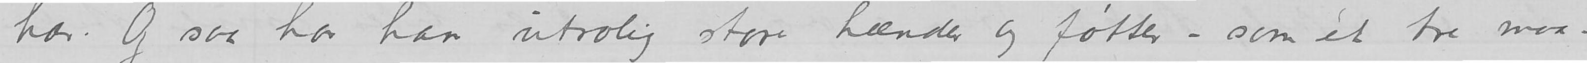har. Og saa har han utrolig store hænder og føtter – som et tre maa-
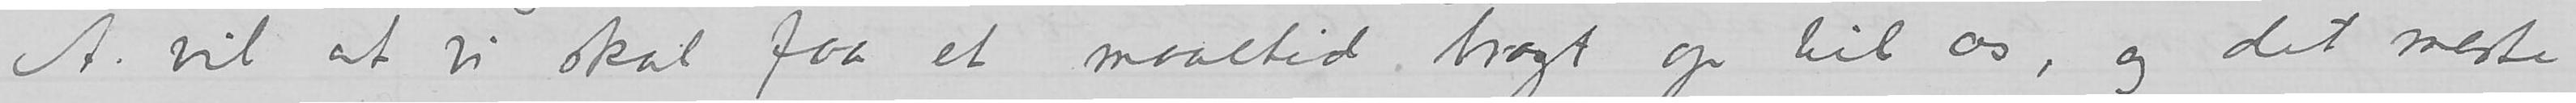A. vil at vi skal faa et maaltid bragt op til os, og det meste
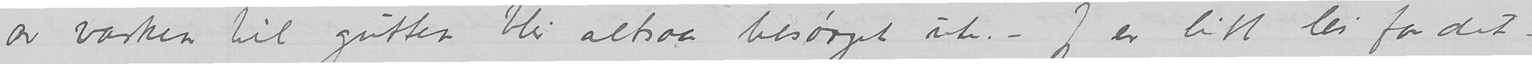av vasken til gutten blir altsaa besørget ute. – Jeg er blitt lei for det
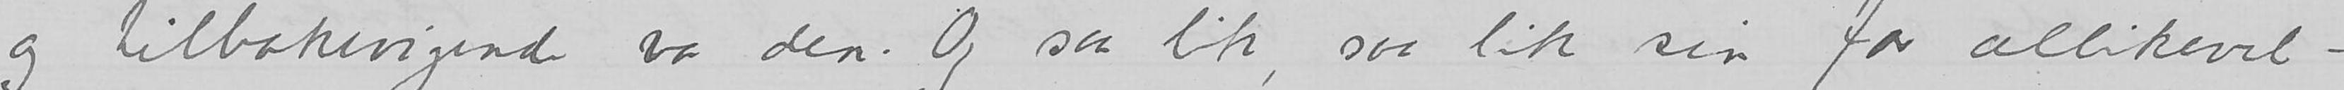og tilbakevigende var den. Og saa lik, saa lik sin far allikevel –.
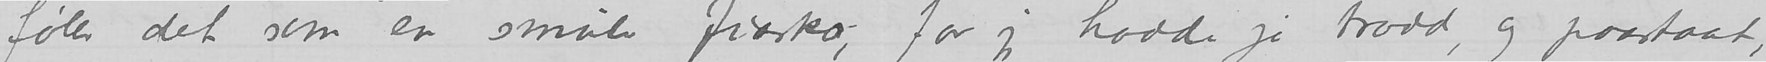–, føler det som en smule fiasko, for jeg hadde jo trodd, og paastaat,
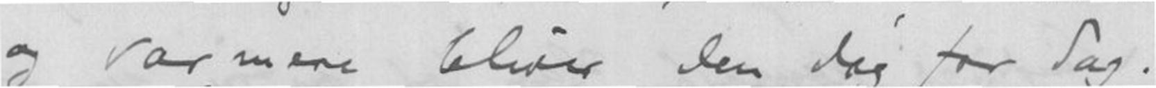og varmere bliver den dag for dag.
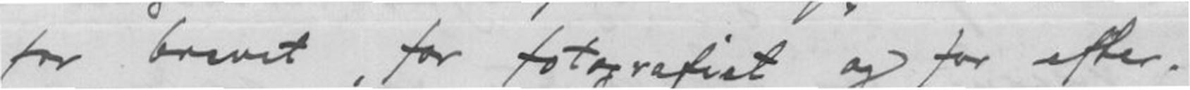for brevet, for fotografiet og for efter-
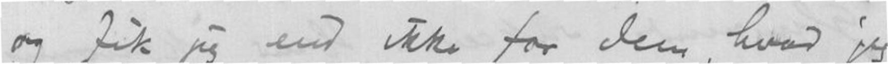og fik jeg end ikke for dem, hvad jeg
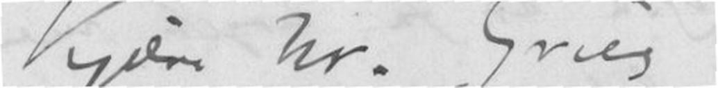Kjære hr. Grieg!
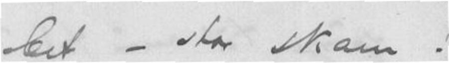bet - stor skam!
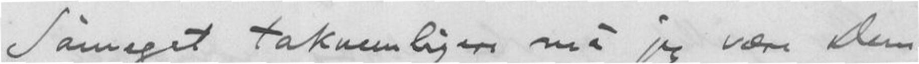Såmeget taknemligere må jeg være Dem
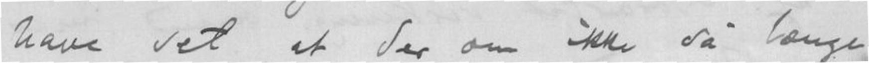have set at der om ikke så længe
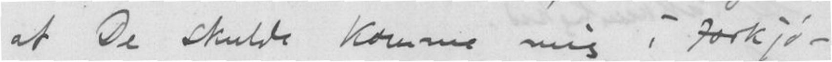at De skulde komme mig i forkjø-
